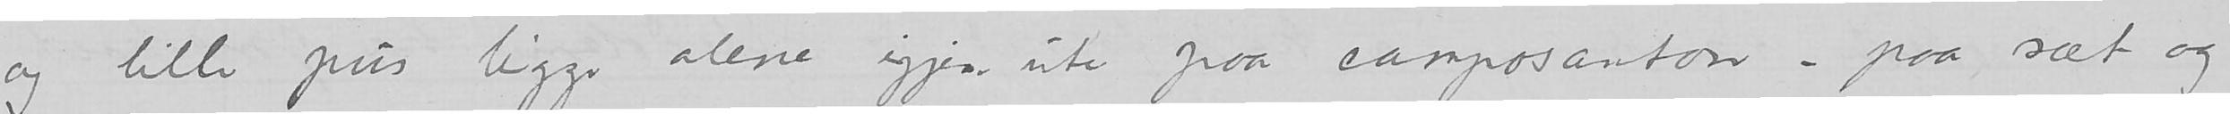og lille pus ligger alene igjen ute paa camposanton – paa sæt og
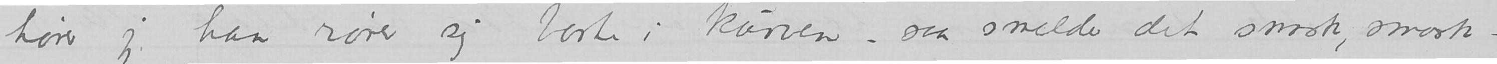hører jeg han rører sig borte i kurven – saa smelder det smask, smask –
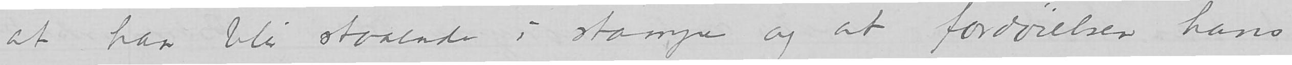at han blir staaende i stampe og at fordøielsen hans
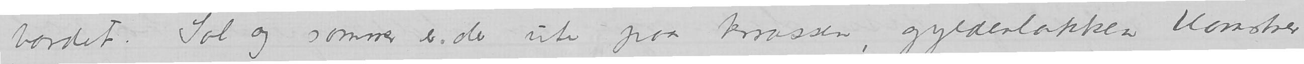bordet. Sol og sommer er der ute paa terrassen, gyldenlakken blomstrer
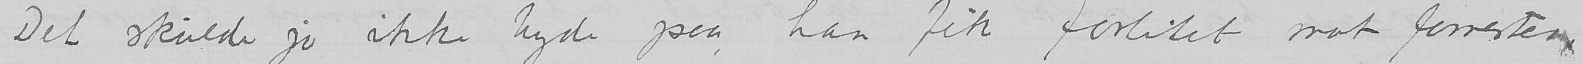Det skulde jo ikke tyde paa, han fik forlitet mat forresten
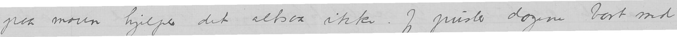paa maven hjelper det altsaa ikke. Jeg pusler dagene bort med
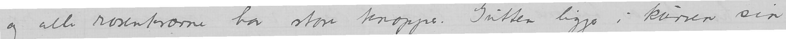og alle rosentrærne har store knopper. Gutten ligger i kurven sin
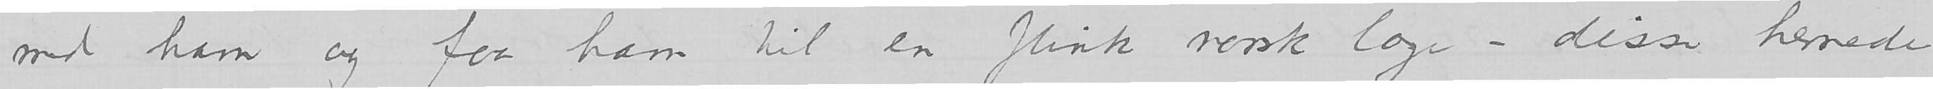med ham og faa ham til en flink norsk læge – disse hernede
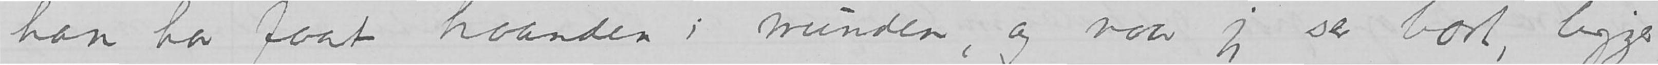han har faat haanden i munden, og naar jeg ser bort, ligger
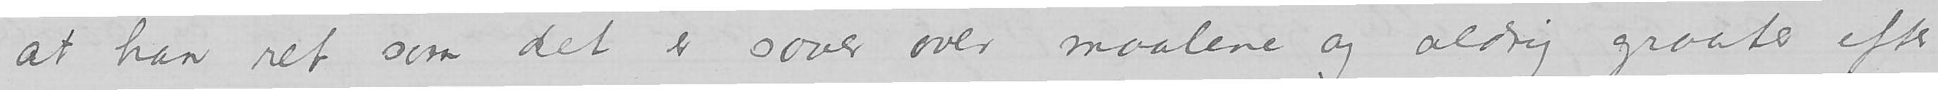at han ret som det er sover over maalene og aldrig graater efter
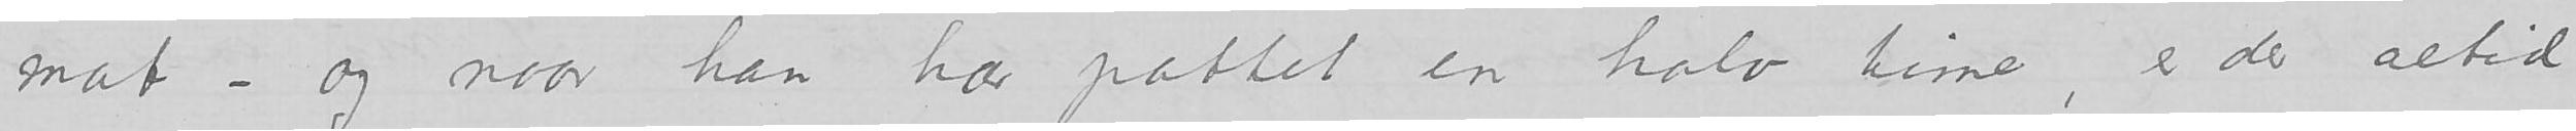mat – og naar han har pattet en halv time, er der altid
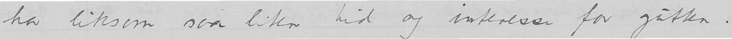har liksom saa liten tid og interesse for gutten.
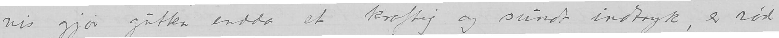vis gjør gutten endda et kraftig og sundt indtryk, er rsød og hvit
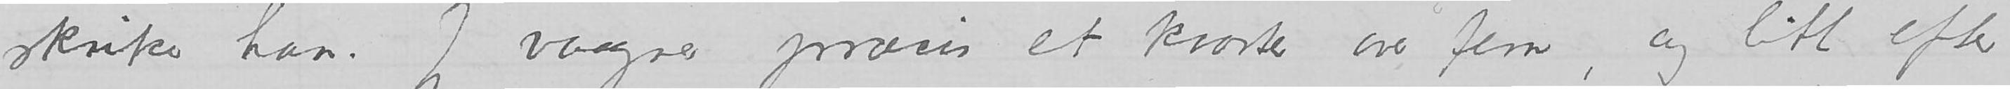skriker han. Jeg vaagner præcis et kvarter over fem, og litt efter
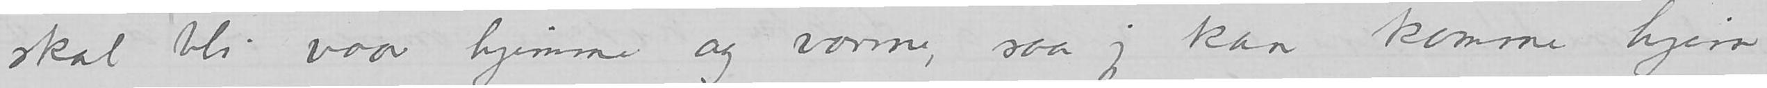skal bli vaar hjemme og varme, saa jeg kan komme hjem
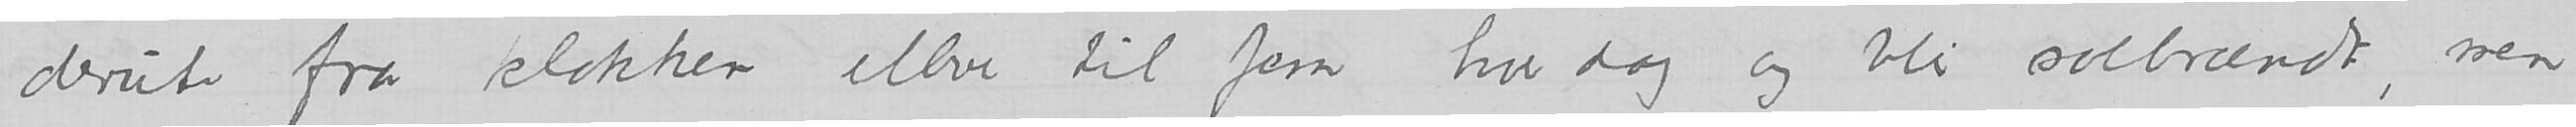derute fra klokken elleve til fem hver dag og blir solbrændt, men
